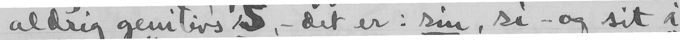aldrig genitivs S, - det er : sin, si - og sit i
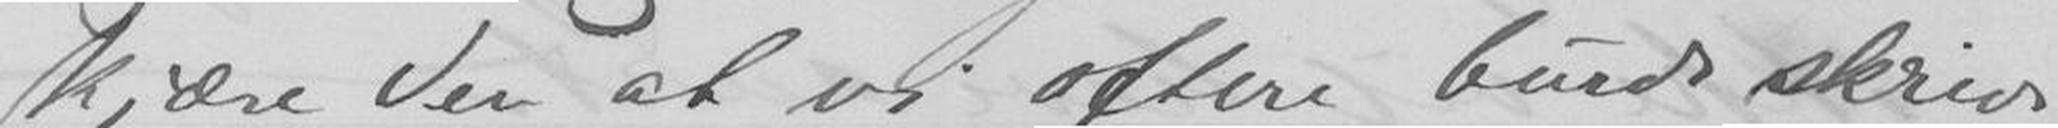kjære Ven at vi oftere burde skrive
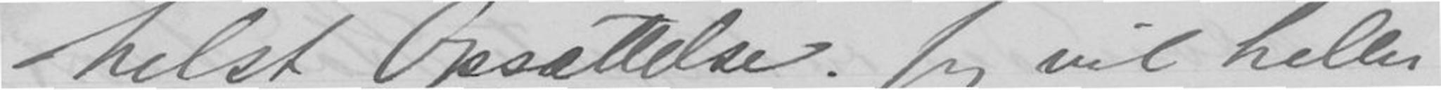helst Opsættelse. Jeg vil heller
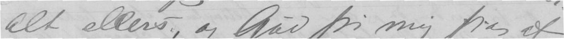alt ellers, og Gud fri mig fra at
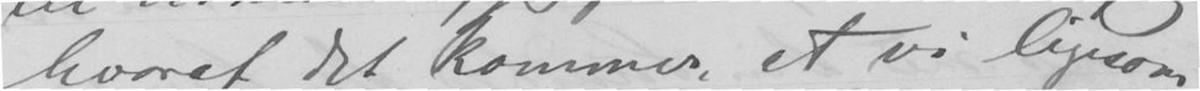hvorfor det kommer at vi ligesom
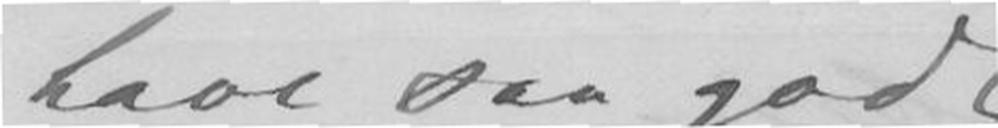have saa godt
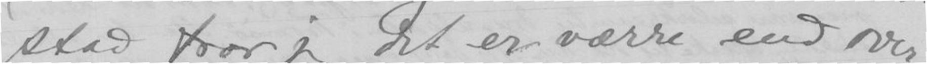stad tror jeg det er værre end over
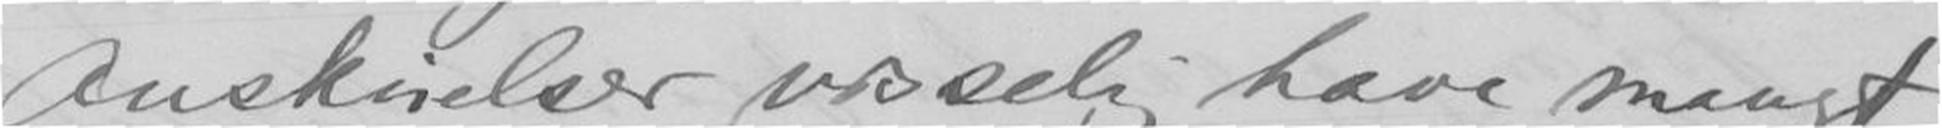Anskuelser visselig have mangt
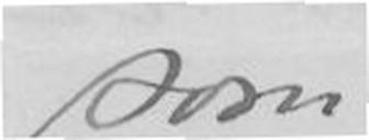som
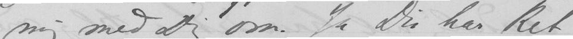mig med Dig om. Ja Du har Ret
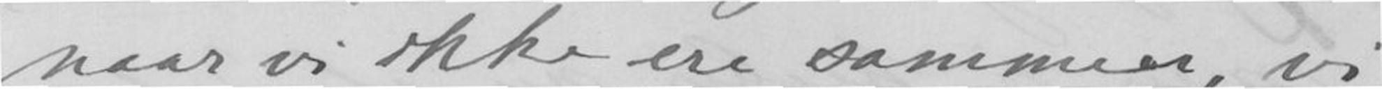naar vi ikke ere sammen, vi
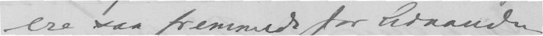ere saa fremmede for hindanden
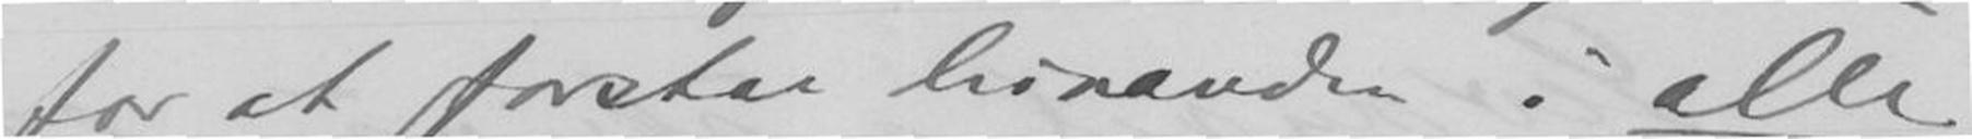for at forstaa hinanden i alle
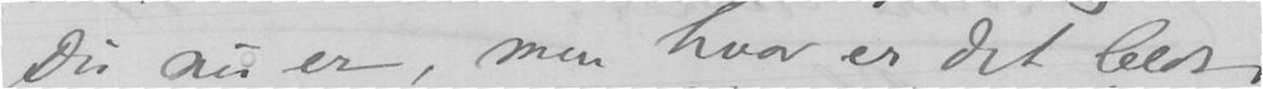der Du nu er, men hvor er det bedre
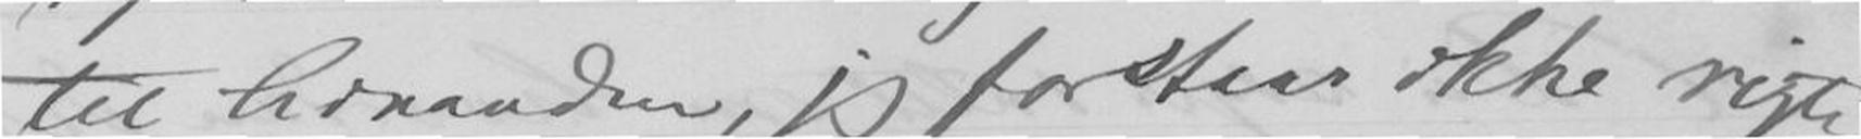til hinanden, jeg forstaar ikke rigtig
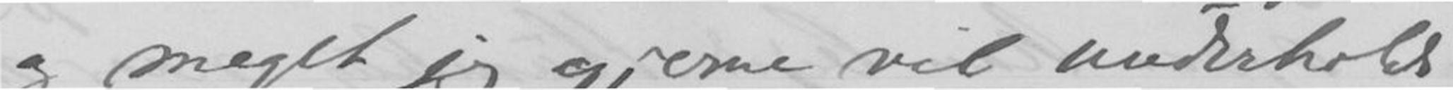og meget jeg gjerne vil underholde
